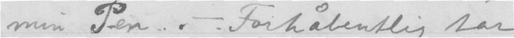min Pen. - Forhåbentlig tar
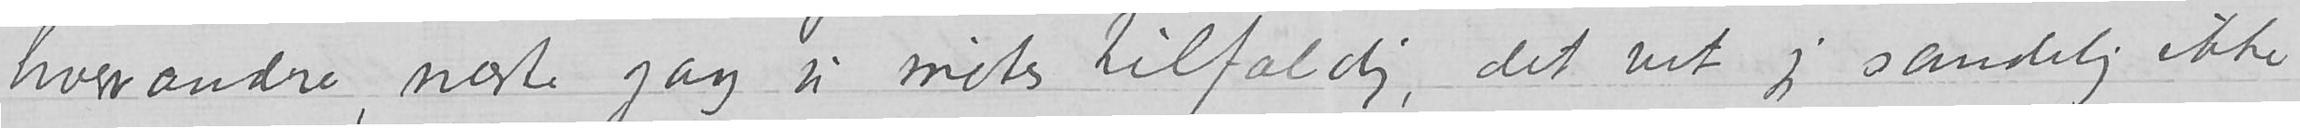hverandre, næste gang vi møtes tilfældig, det vet jeg sandelig ikke,
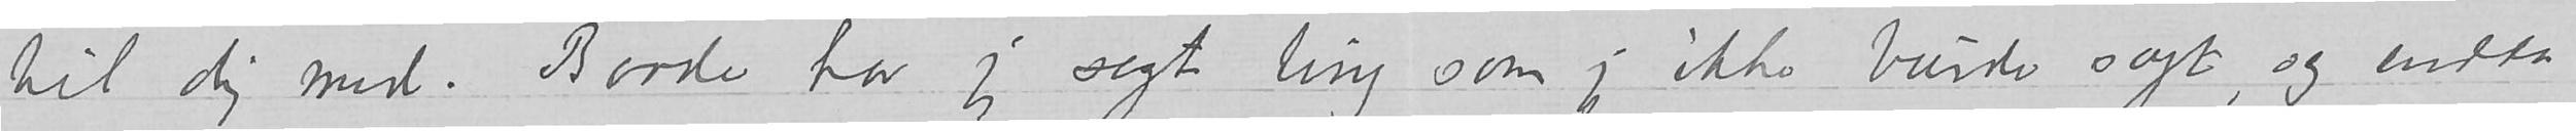til dig med. Baade har jeg sagt ting som jeg ikke burde sagt, og endda
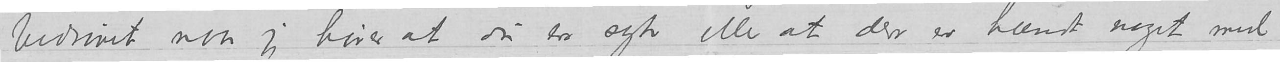bedrøvet naar jeg hører at du er syk eller at der er hændt noget med
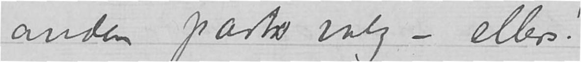anden parts valg - ellers!
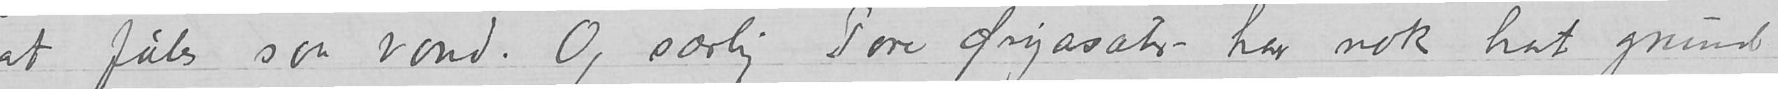at føles saa vond. Og særlig Tore Ørjasæter har nok hat grund
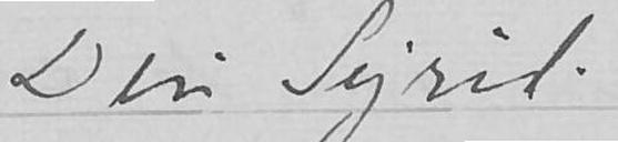Din Sigrid
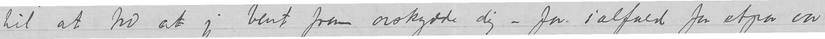til at tro at jeg bent frem avskydde dig - for ialfald for etpar aar
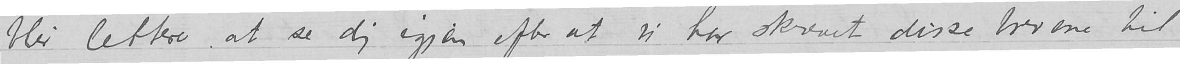blir lettere at se dig igjen efter at vi har skrevet disse brevene til
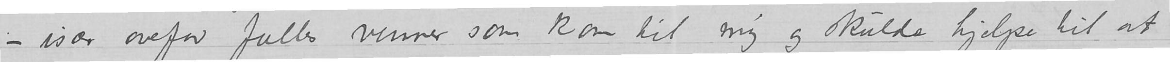især ovefor folks venner som kom til mig og skulde hjelpe til at
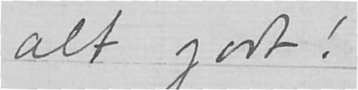alt godt!
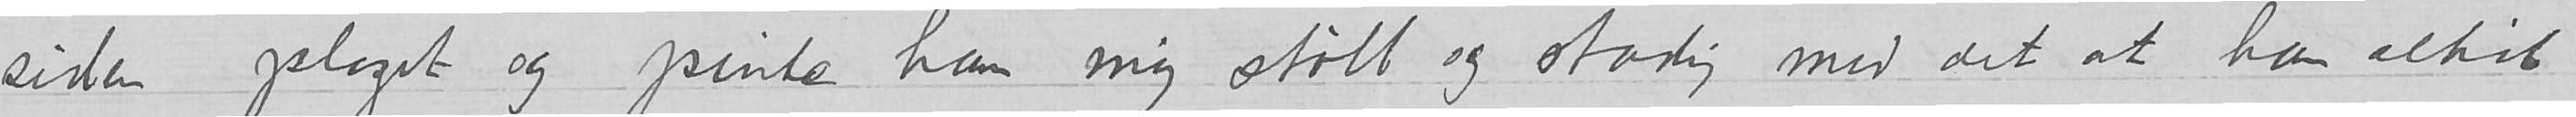siden plaget og pinte han mig støtt og stadig med det at han altid
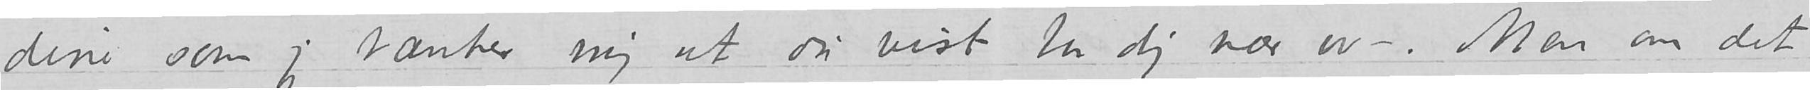dine som jeg tænker mig at du vist tar dig nær av -. Men om det
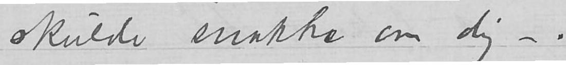skulde snakke om dig -.
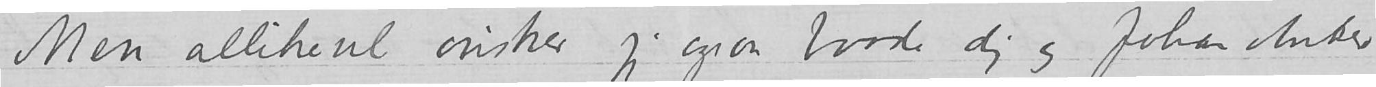Men allikevel ønsker jeg ogsaa baade dig og Johan Anker
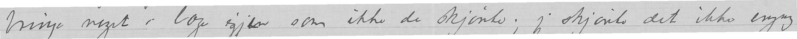bringe noget i lage igjen som ikke de skjønte; jeg skjønte det ikke engang
